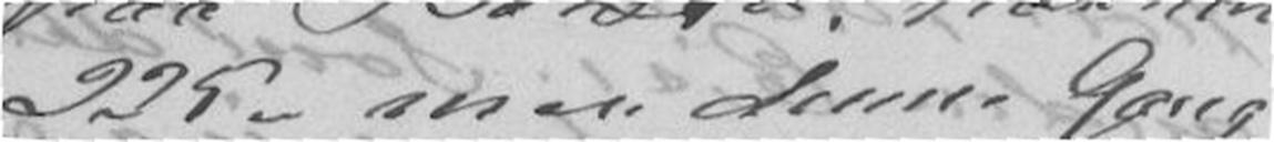235- men denne Gang
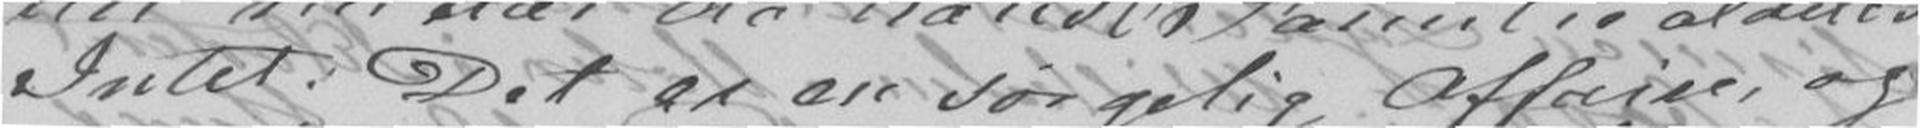Intet. Det er en sørgelig Affaire, og
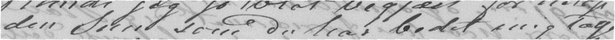den Sum som Du gar badet mig tage
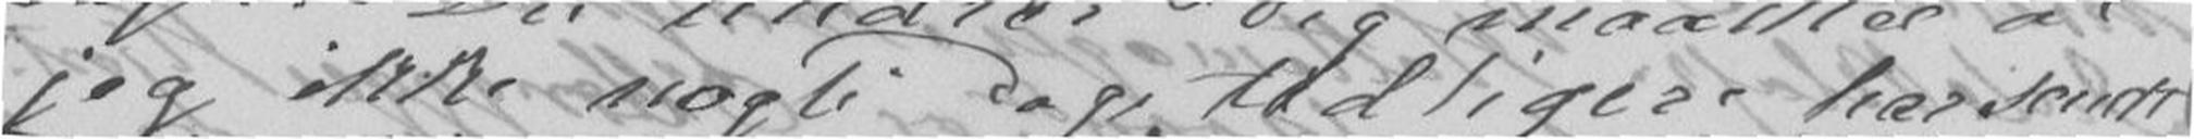jeg ikke nogle Dage tidligere har sendt
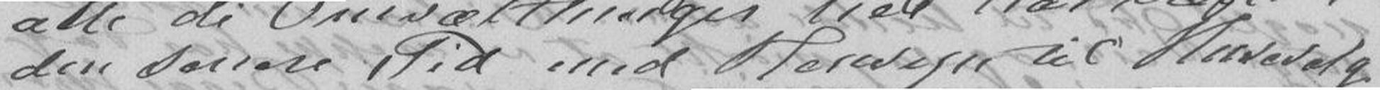den senere Tid med Hensyn til Husesalg
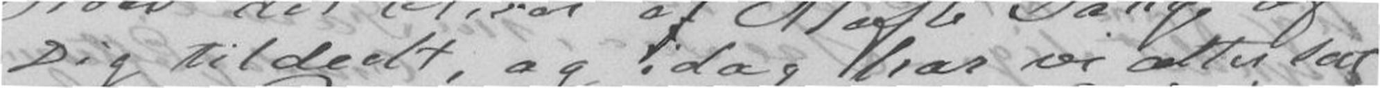Dig tildeelt, og idag har vi atter sat
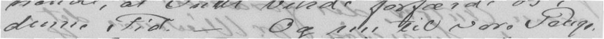denne Tid. - Og nu til vore Penge-
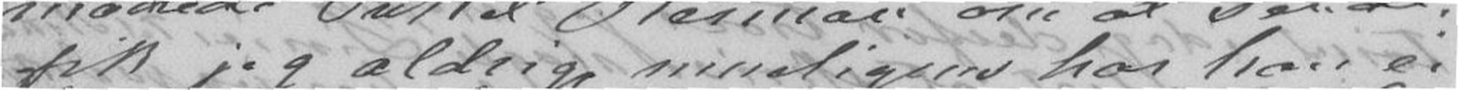fik jeg aldrig, mueligens har han ei
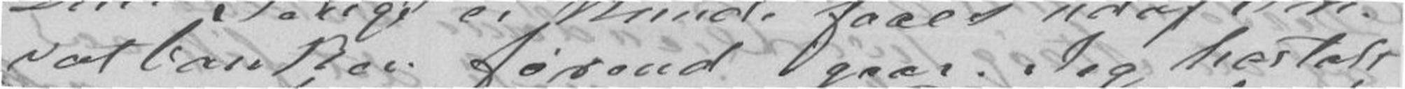vatbanekn førend igaar. Jeg har talt
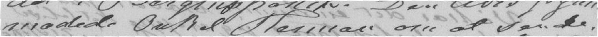modede Onkel Herman om at sende,
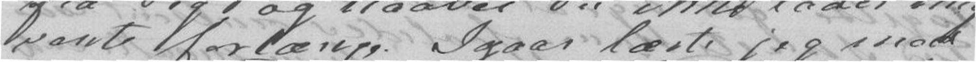vente forlænge. Igaar læste jeg mod
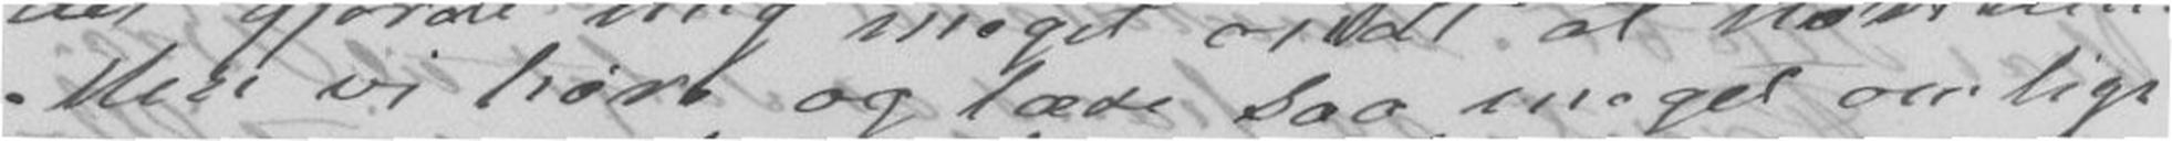Men vi høre og læse saa meget om lig-
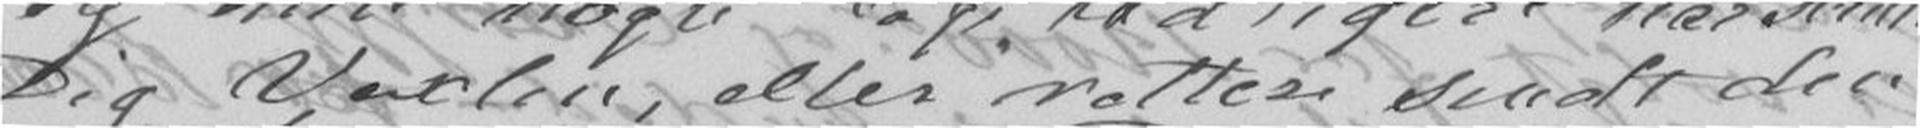Dig Vexlen, eller rettere sendt den
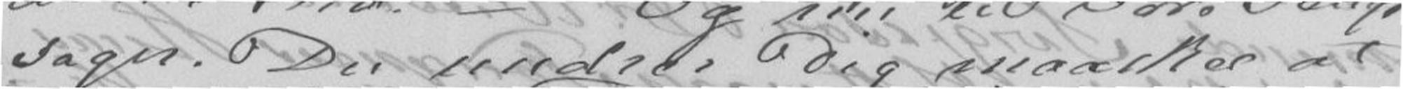sager. Du undrer dig maaskee at
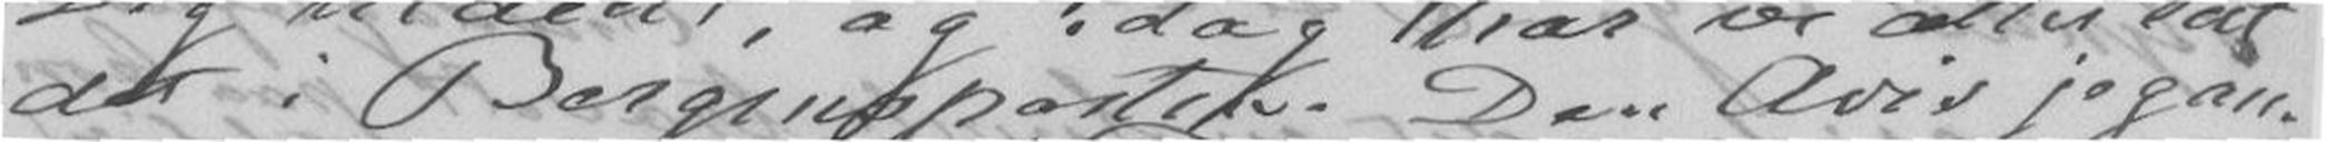det i Bergensposten. Den Avis jeg an-
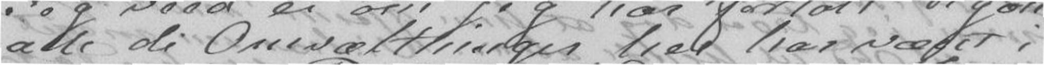alle de Omvæltninger her har været i
